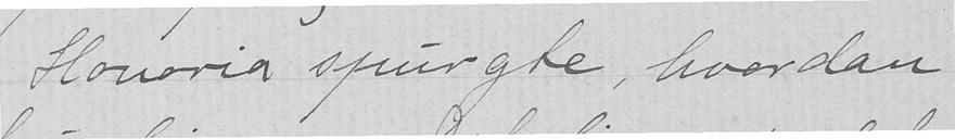Honoria spurgte, hvordan
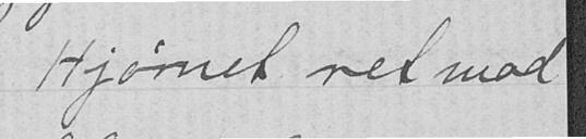Hjørnet ret mod
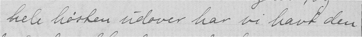hele høsten udover har vi havt den
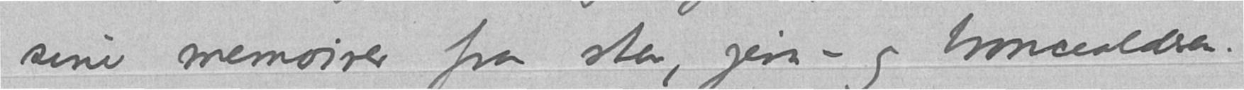sine memoirer fra sten, jern -og broncealderen.
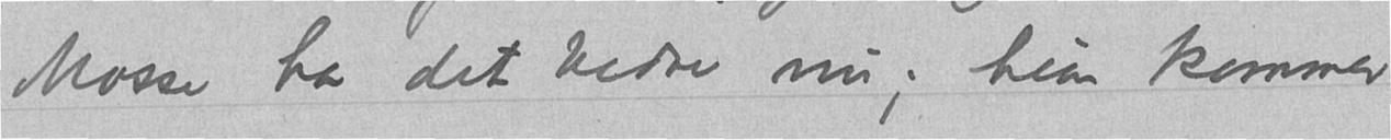Mosse har det bedre nu; hun kommer
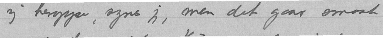sig heroppe, synes jeg, men det gaar smaat
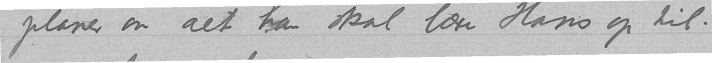planer om alt han skal lære Hans op til.
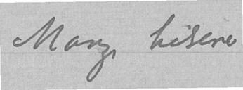Mange hilsener
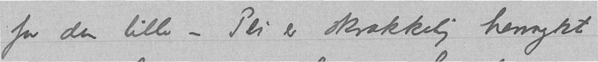for den lille – Pei er skrækkelig henrykt
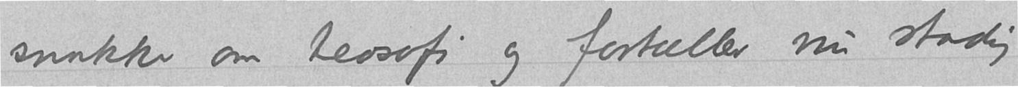snakke om teosofi og fortæller nu stadig
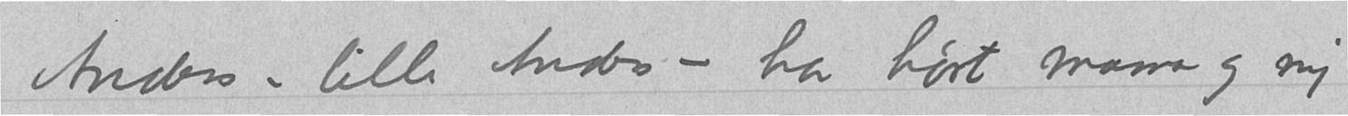Anders – lille Anders – har hørt mama og mig
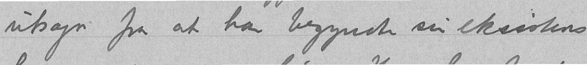utsagn fra at han begyndte sin eksistens
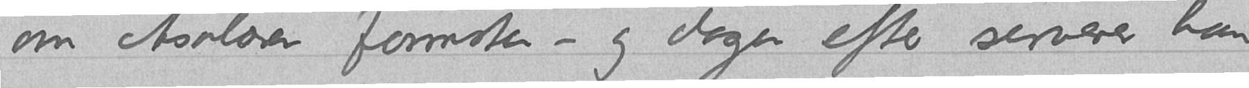om Asalæren forresten – og dagen efter serverer han
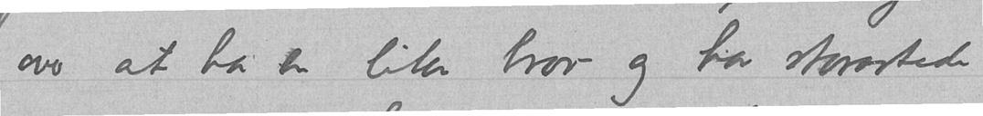over at ha en liten bror og har storartede
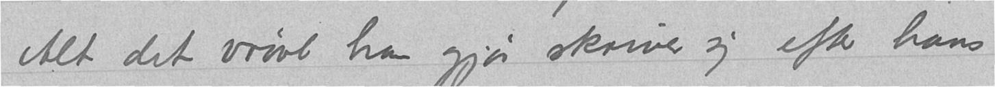Alt det vrøvl han gjør skriver sig efter hans
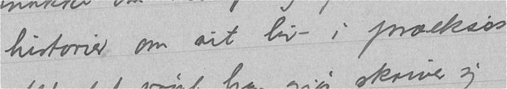historier om sit liv i præeksistenserne.
